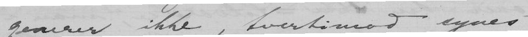generer ikke, tvertimod synes
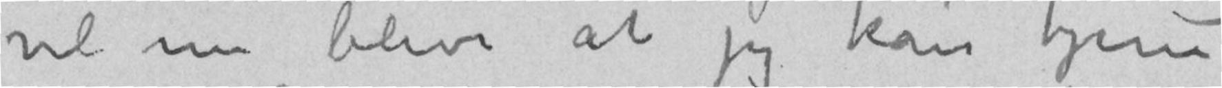vil nu blive at jeg kan tjene
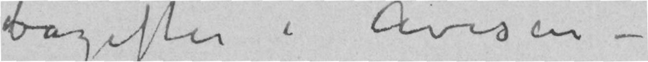bagefter i Avisen –
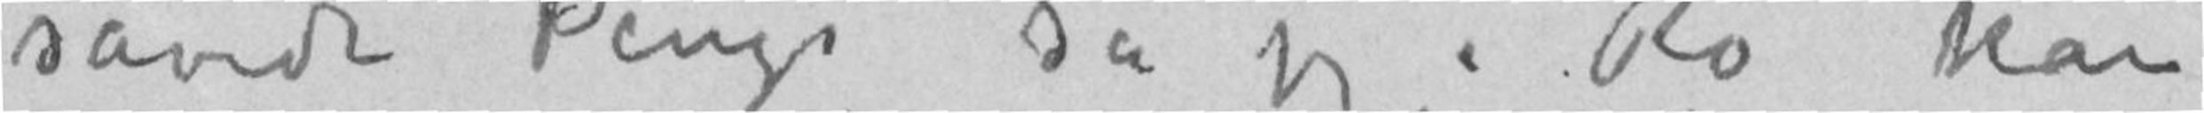såvidt Penge så jeg i Ro kan
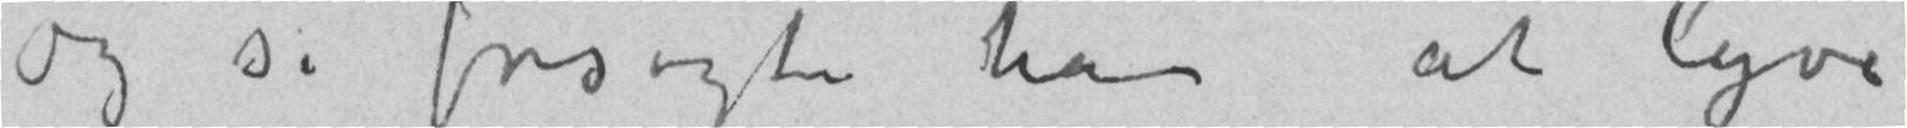og så forsøgte han at lyve
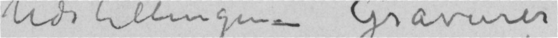Udstillingen – Gravurer
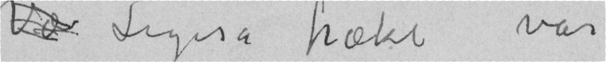Væ Ligeså frækt var
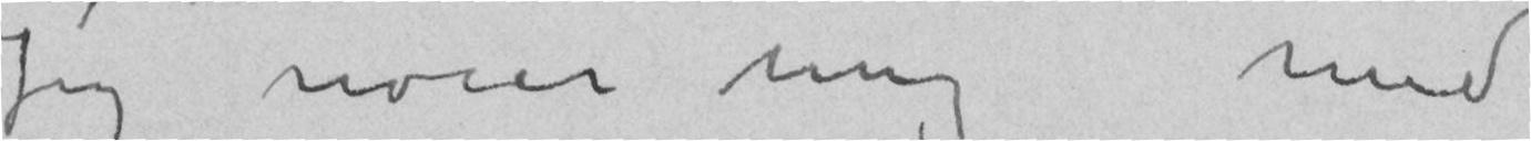Jeg nøier mig med
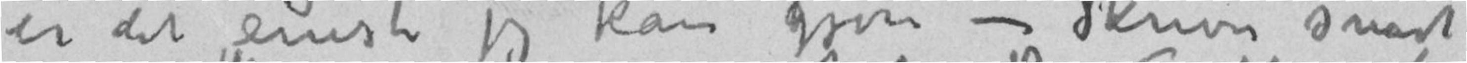er det eneste jeg kan gjøre – Skriver snart
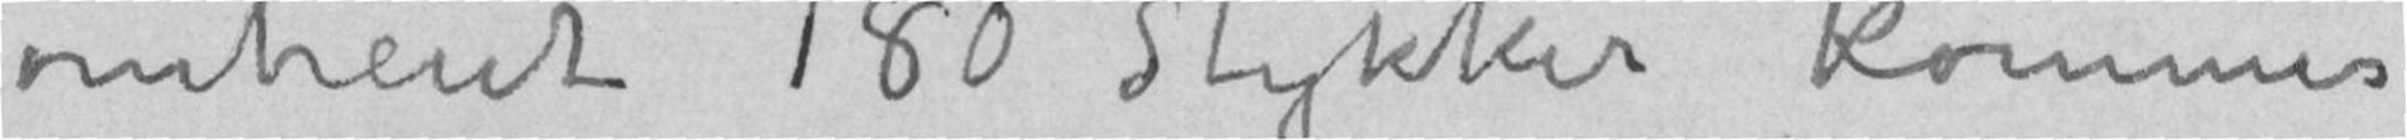omtrent 180 Stykker kommer
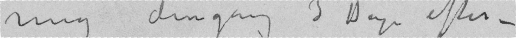mig dengang 3 Dage efter –
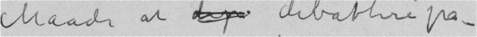Maade at dep debattere på –
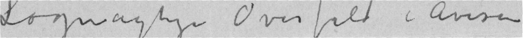Løgnaktige Overfald i Aviser
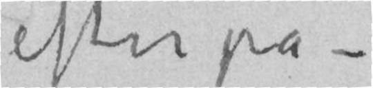efterpå –
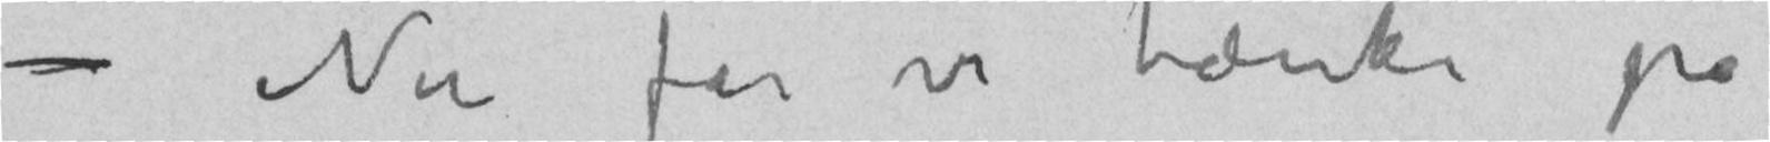– Nu får vi tænke på
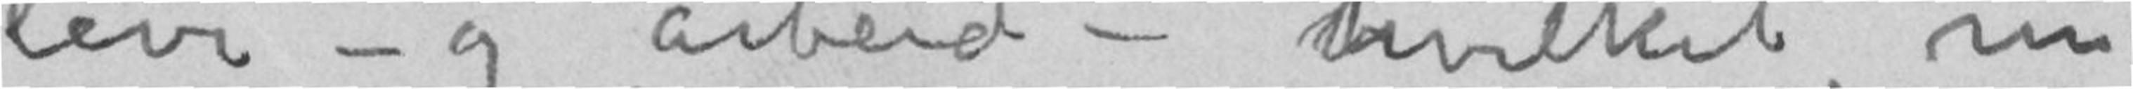leve – og arbeide – hvilket nu
